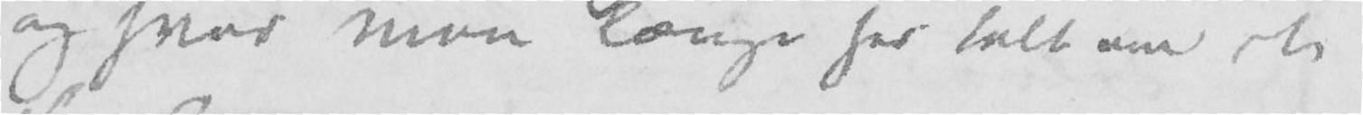og hvor man længe har talt om et
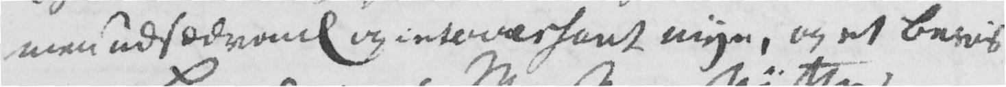men udsædvanl og interessant vye, og et bevis
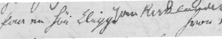paa en høi Klippe paa Kirklandet
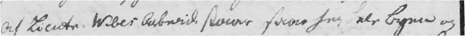af Lieutn. Wibes Abeide staaer saae jeg hele Byen og
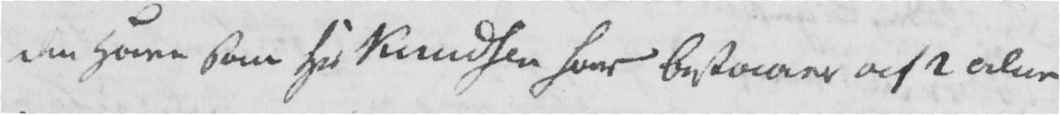Den Have som Hr Knudsen har bestaaer af 2 alen
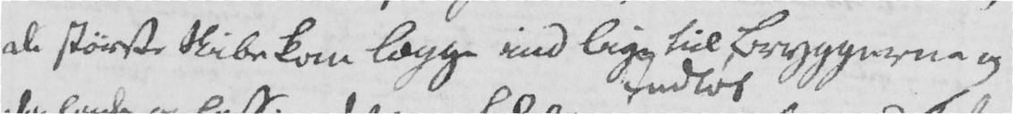de største Skibe kan lægge ind lige til Bryggerne og
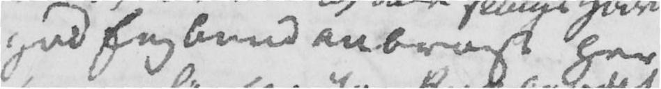god Engbund anbragt her
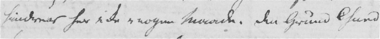hindrer her icke i nogen Maade. Den Grund Csund
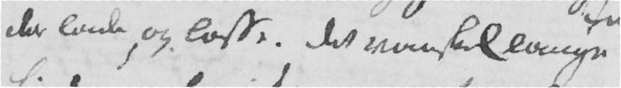der lade og laste. Det vanskel lange
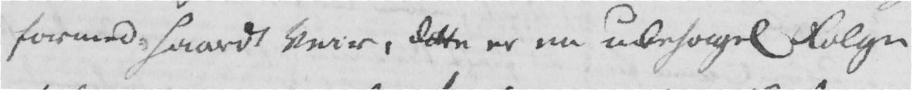formed haardt Veir, dette er en ubehagel Følge
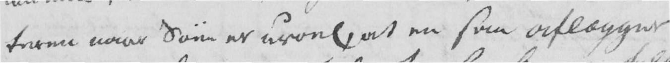teren naar Søen er uroel, at en som aflægger
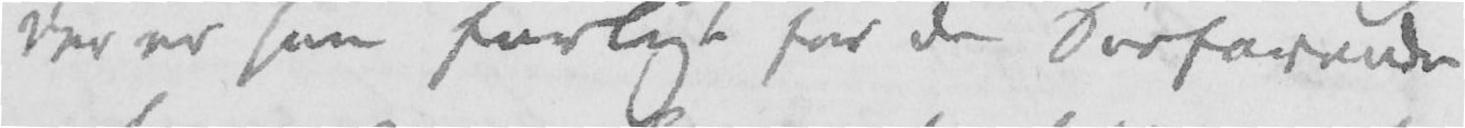der er saa farligt for de Søefarende
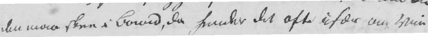en maa skee i Baad, da hender det ofte især om Vin-
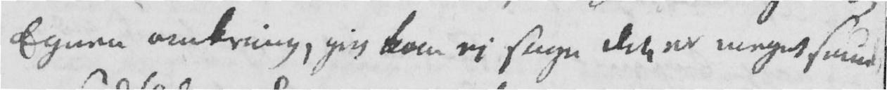Egnen omkring, jeg kan ej sige det er meget smuckt,
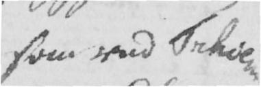som ved Trhiem
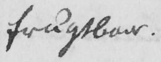frugtbar.
